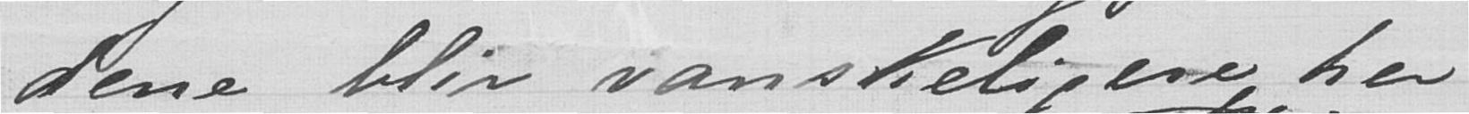dene blir vanskeligere her
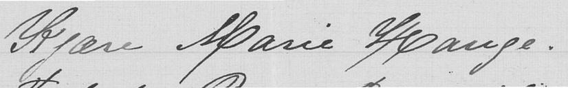Kjære Marie Hauge.
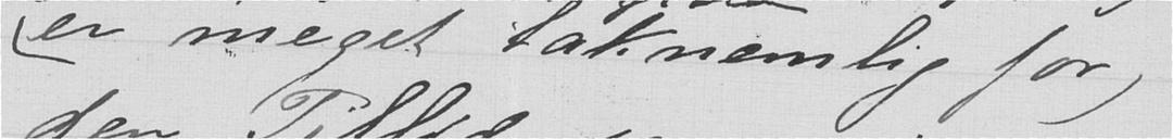er meget taknemlig for,
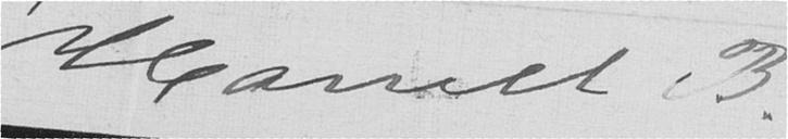Harriet B.
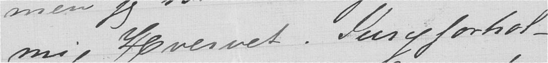mig Hvervet. Juryforhol-
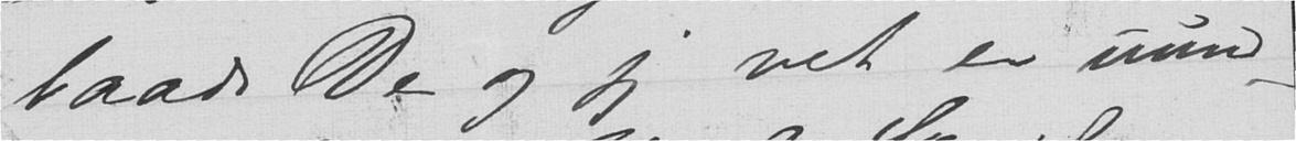baade De og jeg vet er uund-
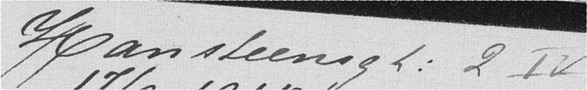Hansteensgt. 2 IV
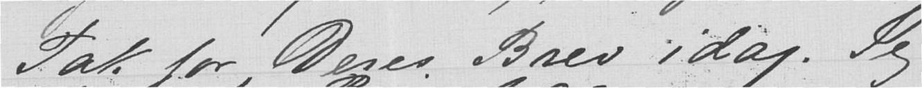Tak for Deres Brev idag. Jeg
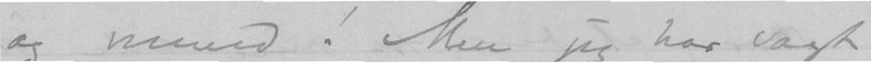og mund! Men jeg har sagt
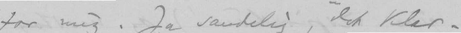for mig. Ja sandelig, det klar-
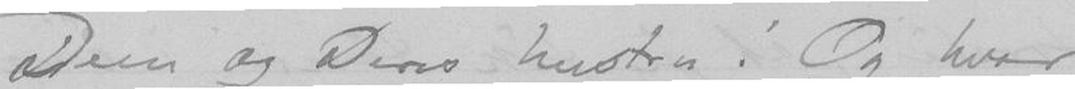Dem og Deres hustru! Og hvor
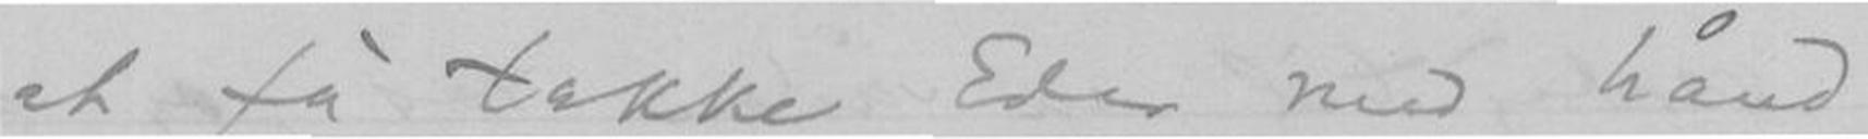at få takke Eder med hånd
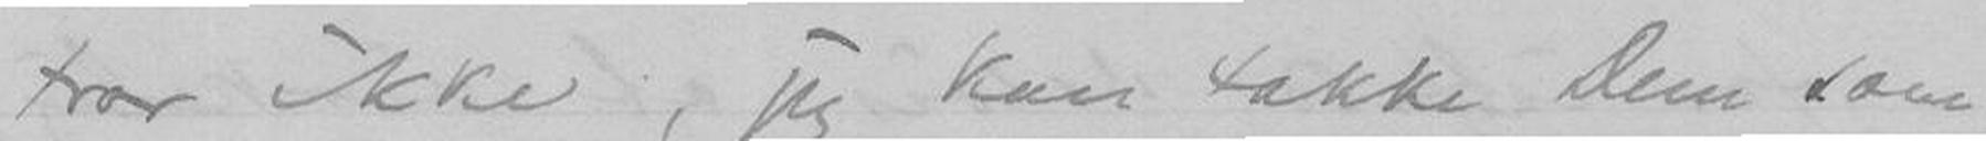tror ikke, jeg kan takke Dem som
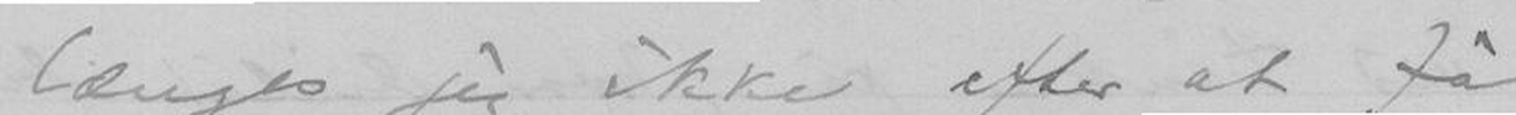længes jeg ikke efter at få
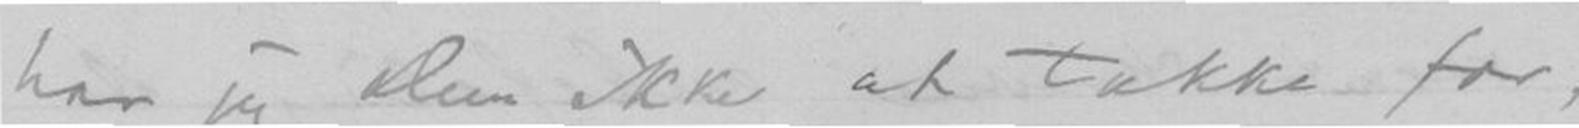har jeg Dem ikke at takke for,
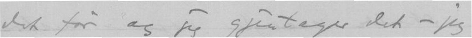det før og jeg gjentager det - jeg
